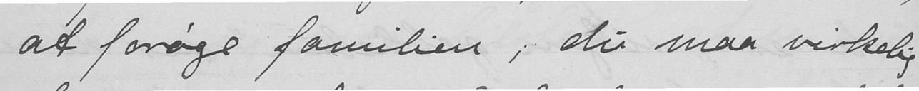at forøge familien; du maa virkelig
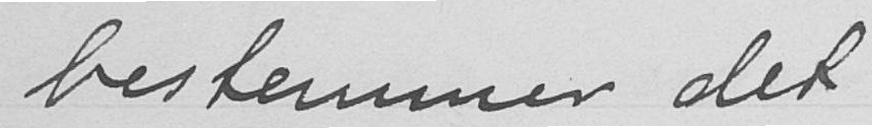bestemmer det.
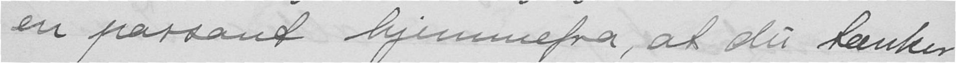en parsant hjemmefra, at du tænker
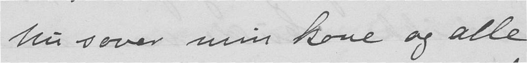Nu sover min kone og alle
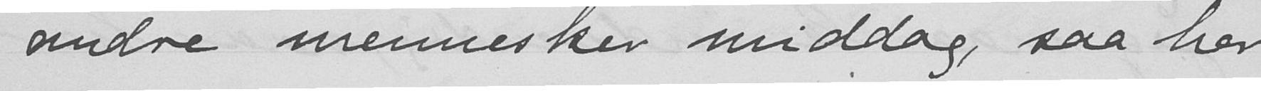andre mennesker middag saa her
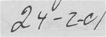24-201
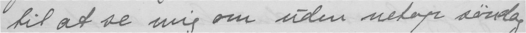til at se mig om uden netop søndag
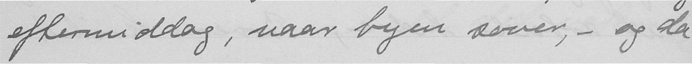eftermiddag, naar byen sover, - og da
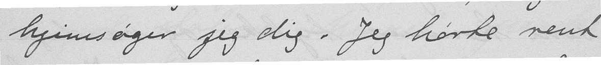hjemsøger jeg dig. Jeg hørte rent
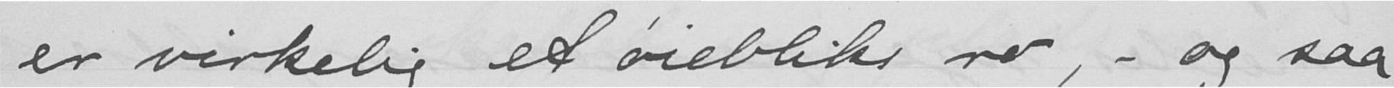er virkelig et øiebliks ro, - og saa
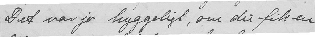Det var jo hyggeligt, om du fik en
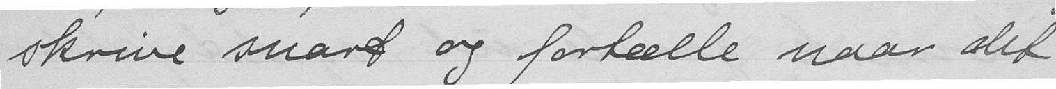skrive snart og fortælle naar det
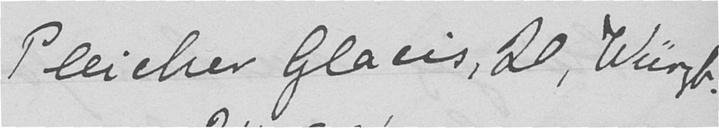Pleicher Glacis, 20, Wrk
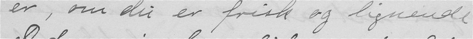er, om du er frisk og lignende.
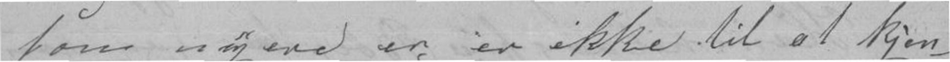som nyere er er ikke til at kjen-
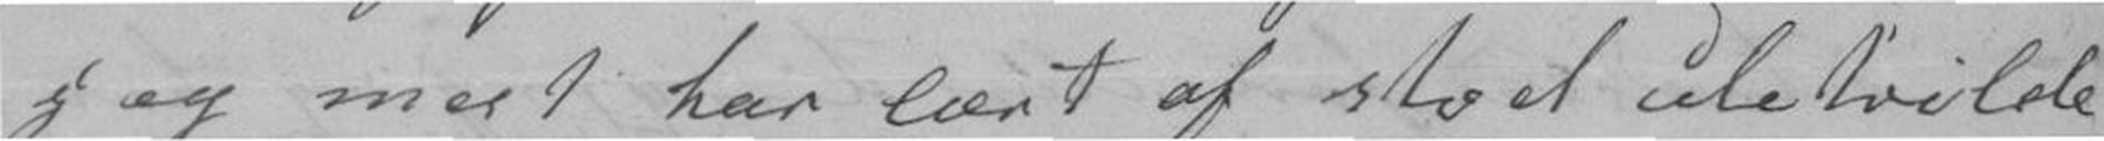jeg mest har lært af stod udetvilde
